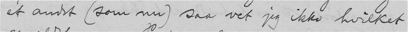et andet (som nu) saa vet jeg ikke hvilket
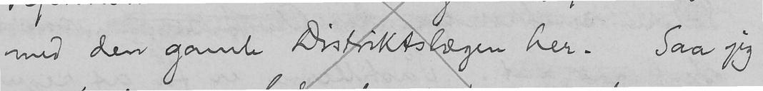med den gamle Distriktslægen her. Saa jeg
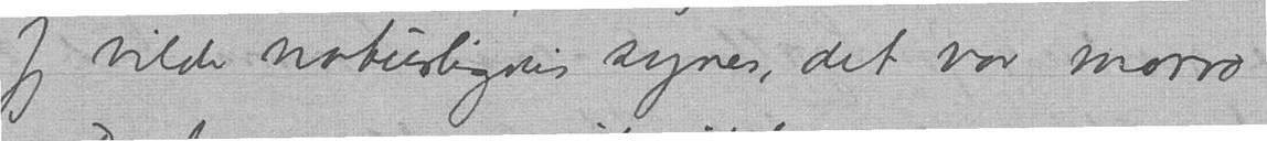Jeg vilde naturligvis synes, det var morro
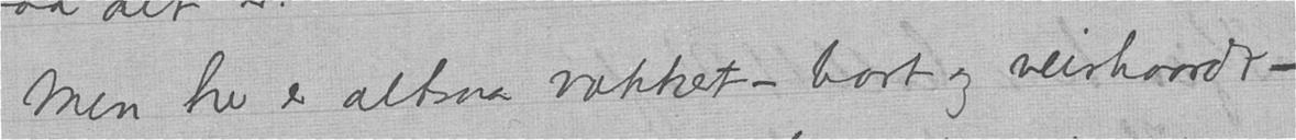Men her er altsaa vakkert – bart og veirhaardt -
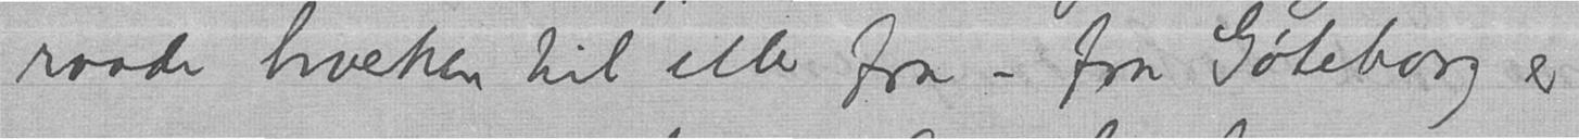raade hverken til eller fra – fra Gøteborg er
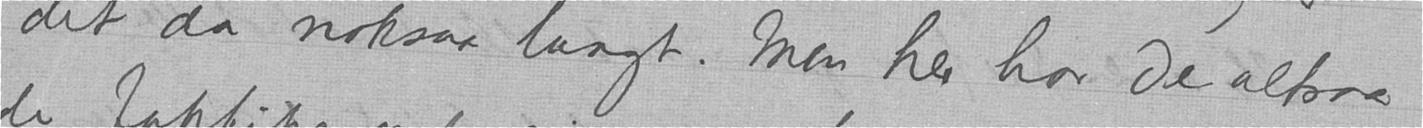det da noksaa langt. Men her har De altsaa
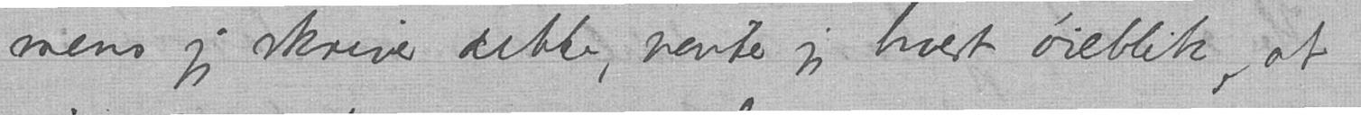mens jeg skriver dette, venter jeg hvert øieblik, at
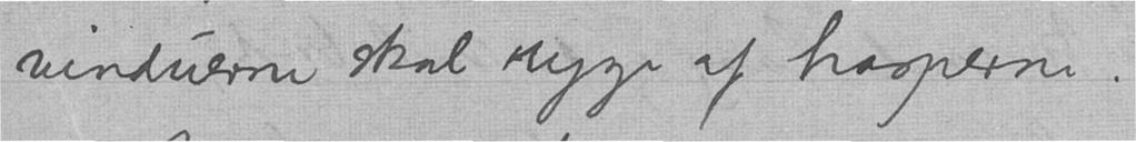vinduerne skal ryge af hasperne.
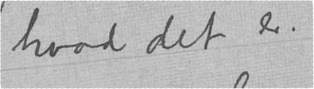hvad det er.
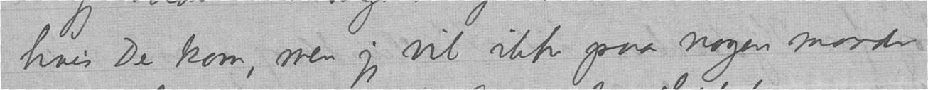hvis De kom, men jeg vil ikke paa nogen maade
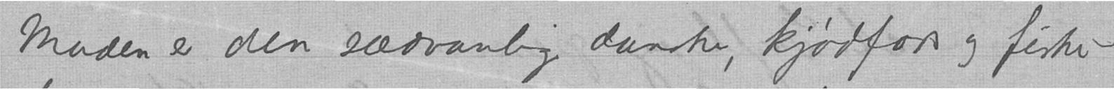Maden er den sædvanlig danske, kjødfars og fiske-
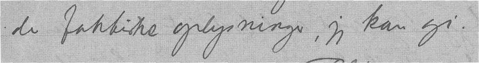de faktiske oplysninger, jeg kan gi.
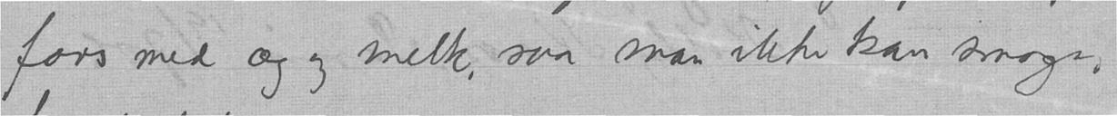fars med æg og melk, saa man ikke kan smage,
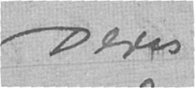Deres
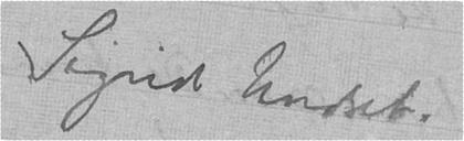Sigrid Undset.
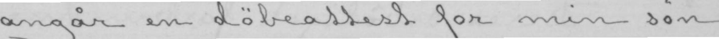angår en døbeattest for min søn
